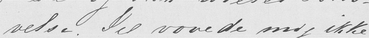velse. Jeg vovede mig ikke
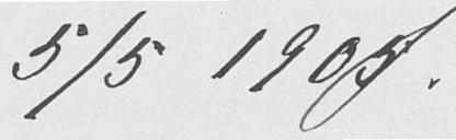5/5 1905.
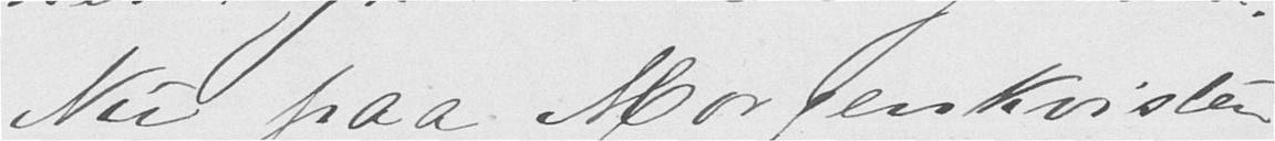Nu paa Morgenkvisten
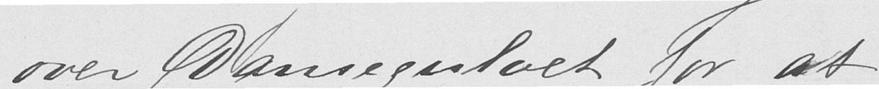over Dansegulvet for at
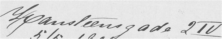Hansteensgade 2 IV
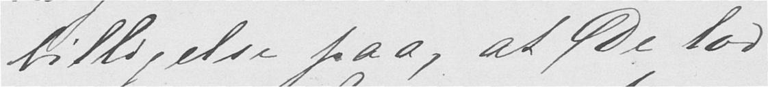billigelse paa, at De lod
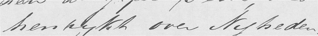henrykt over Nyheden.
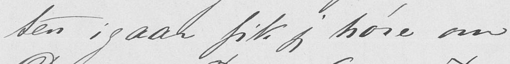ten igaar fik jeg høre om
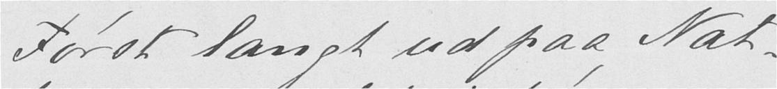Først langt udpaa Nat-
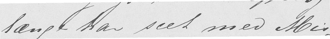længe har seet med Mis-
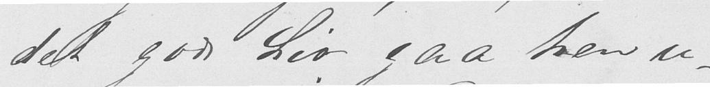det gode Liv gaa hen u-
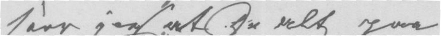seer jeg at De alt paa
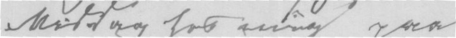Middag hos mig paa
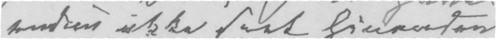endnu ikke seet hinanden
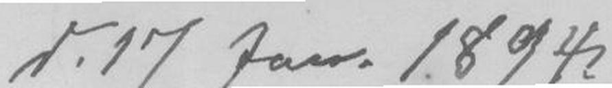d. 17. Jan. 1894
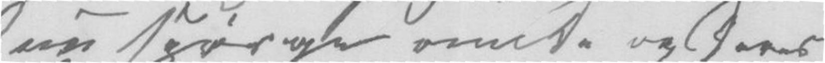nu spørge om De og Deres
